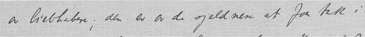av liebhabere; den er av de sjeldnere at faa tak i.
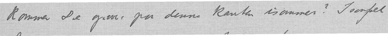kommer De opover paa denne kanten isommer? Isaafal
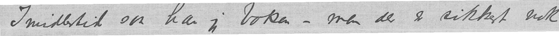Imidlertid saa har jeg boken - men der er sikkert nok
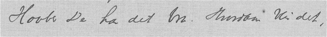Haaber De har det bra. Hvordan blir det,
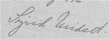Sigrid Undset.
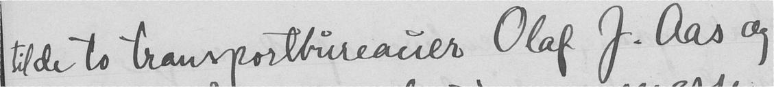til de to transportbureauer Olaf J. Aas og
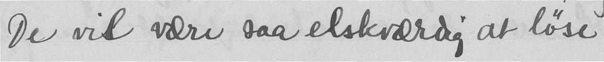De vil være saa elskværdig at løse
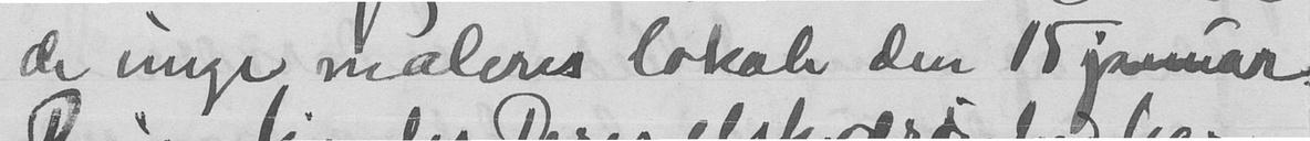de unge maleres lokale den 15 januar.
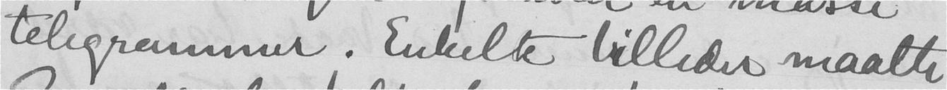telegremmer. Enkelte billeder maatte
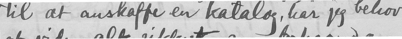til at anskaffe en katalog, har jeg behov
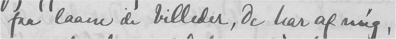faa laane de billeder, De har af mig,
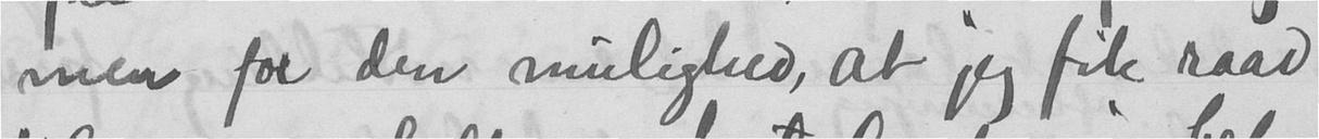men for den mulighed, at jeg fik raad
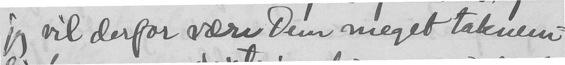jeg vil derfor være Dem meget taknem-
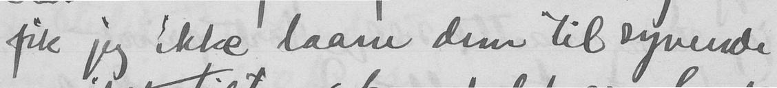fik jeg ikke laane dem til syvende
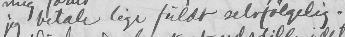jeg betale lige fuldt selvfølgelig.
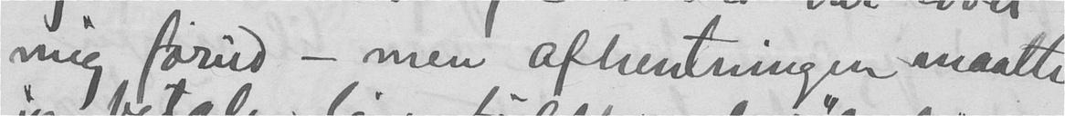mig forud - men afhentningen maatte
